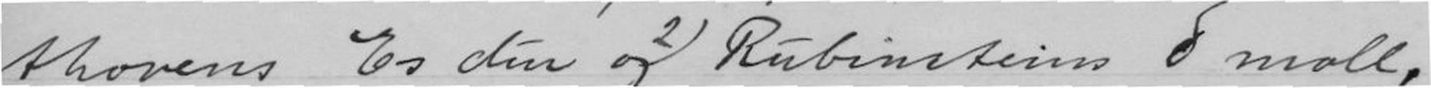thovens Es dur og 2)Rubinsteins d moll,
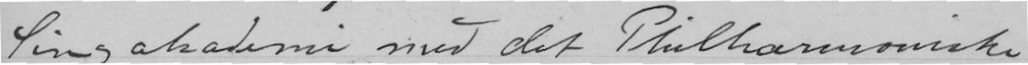Singakademi med det Philharmoniske
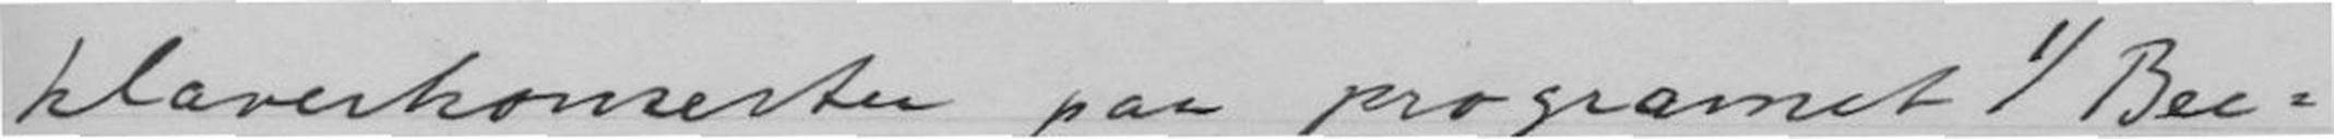klaverkonserter paa programmet 1) Bee-
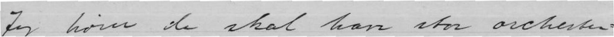Jeg hører de skal have stor orchester-
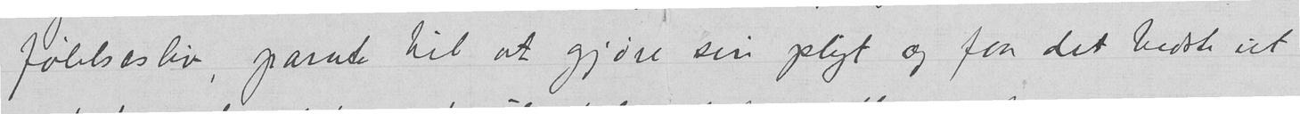følelsesliv, parate til at gjøre sin pligt og faa det bedste ut
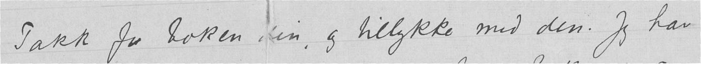Takk for boken den, og tillykke med den. Jeg har
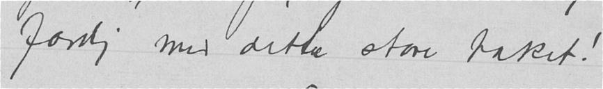færdig med dette store taket!
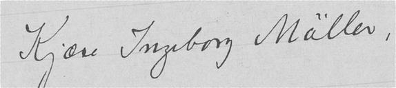Kjære Ingeborg Møller,
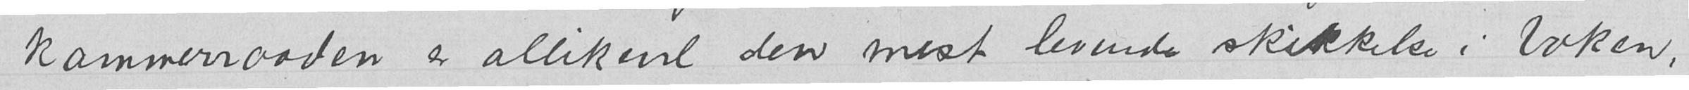kammerraaden er allikevel den mest levende skikkelse i boken,
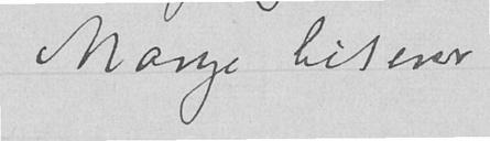Mange hilsener
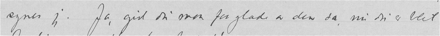synes jeg. Ja, gid du maa faa glæde av den da, nu du er blit
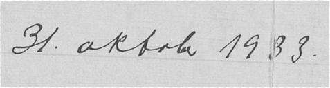31. oktober 1933.
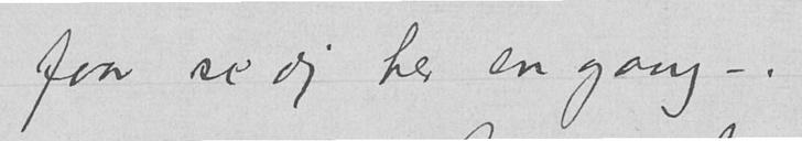jeg faar se dig her en gang –.
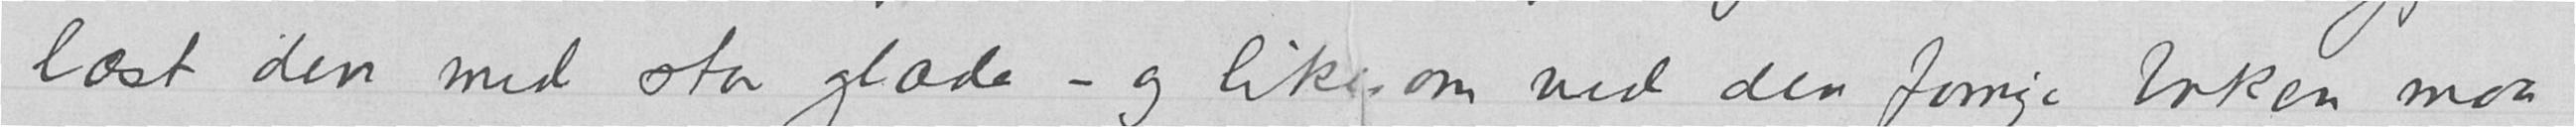læst den med stor glæde - og likesom ved den forrige boken maa
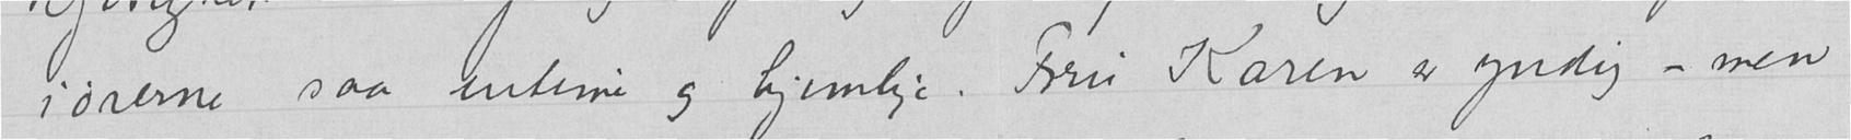iørerne saa intime og hjemlige. Fru Karen er yndig - men
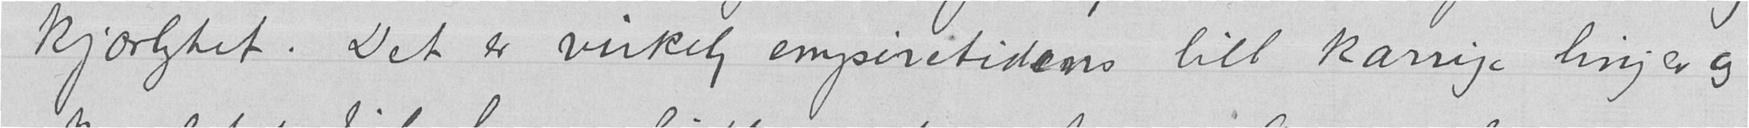kjærlighet. Det er virkelig empiritidens litt karrige linjer og
